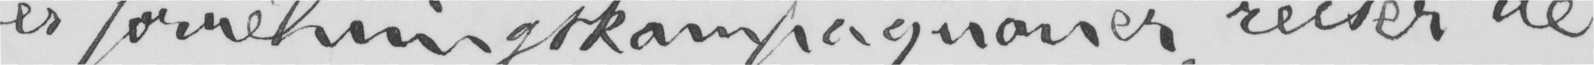er forretningskompagnoner, reiser de
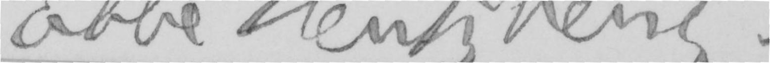Ebbe Hertzberg.
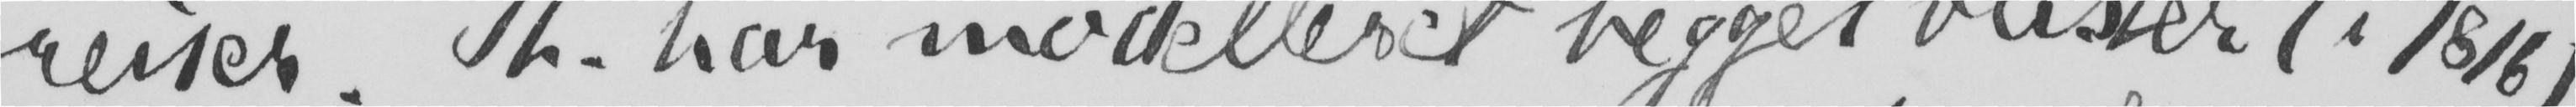reiser. Th. har modelleret begges buster (i 1816),
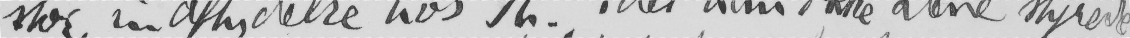stor indflydelse hos Th., idet han ikke alene styrede
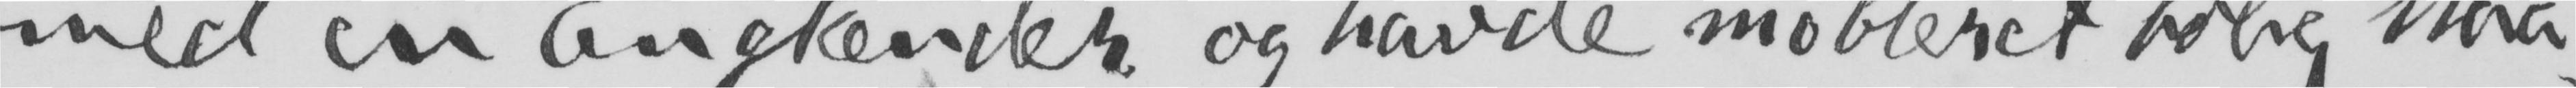med en Englænder og havde møbleret bolig staa-
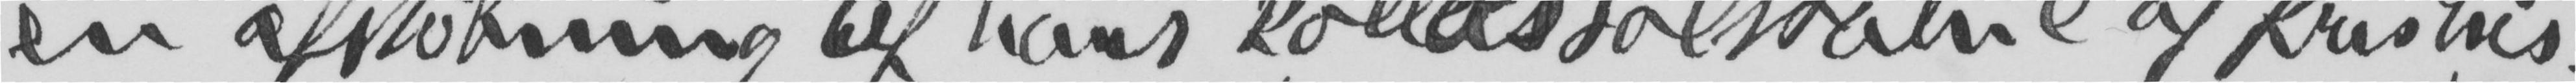en afstøbning af hans kollossalstatue af Kristus;
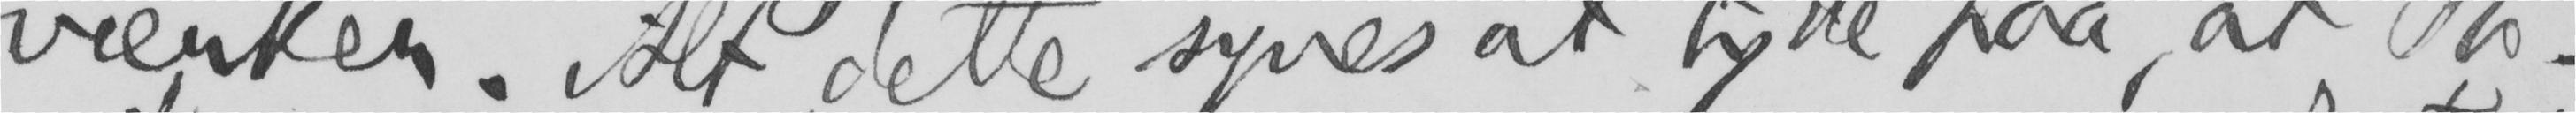værker. Alt dette synes at tyde paa, at Th.
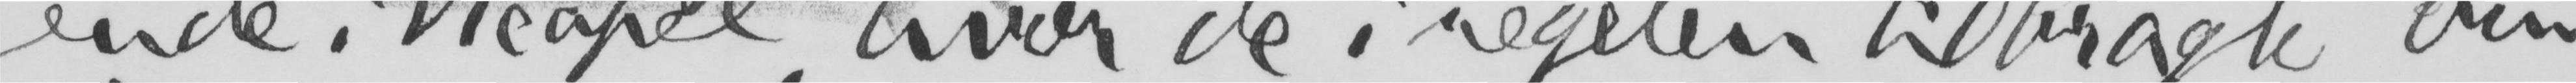ende i Neapel, hvor de i regelen tilbragte vin-
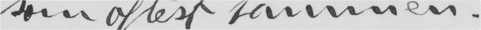som oftest sammen.
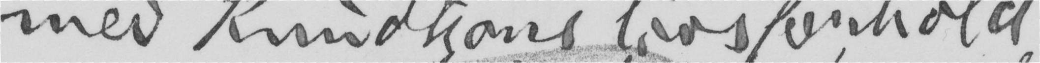med Knudtzons livsforhold.
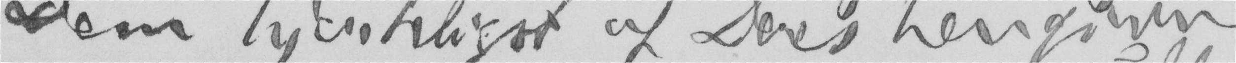dem hjerteligst af Deres hengivne
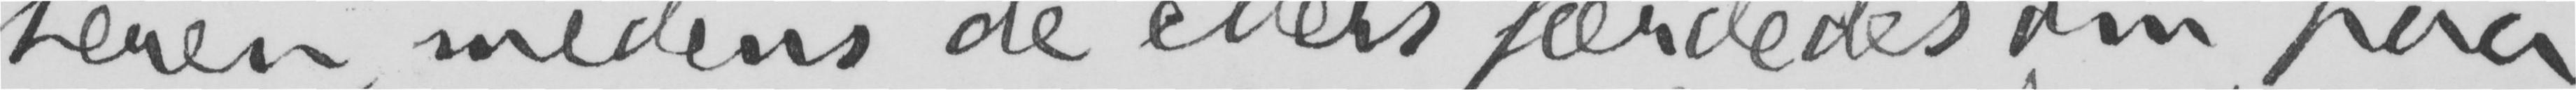teren, medens de ellers færdedes om paa
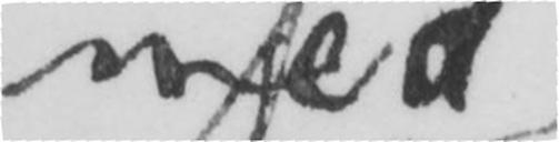med
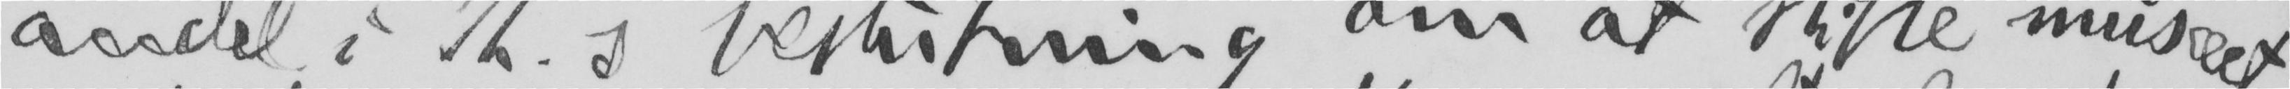andel i Th.’s beslutning om at stifte musæet
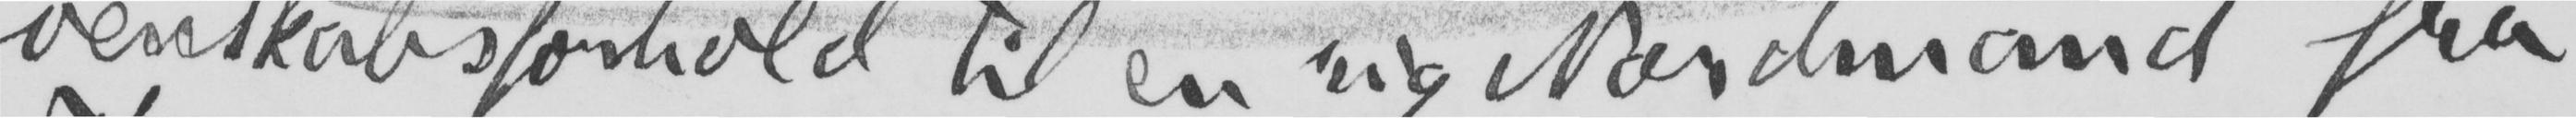venskabsforhold til en rig Nordmand fra
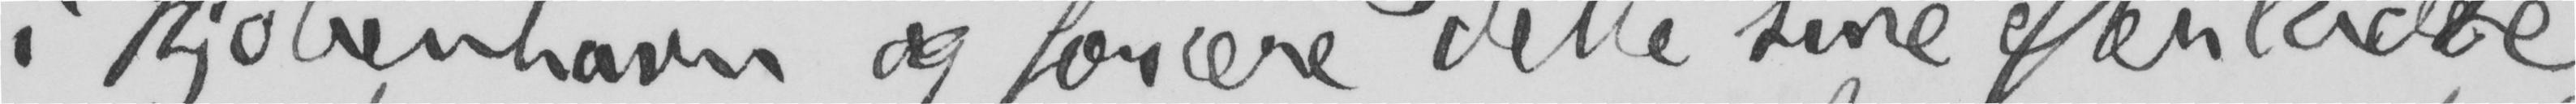i Kjøbenhavn og forære dette sine efterladte
